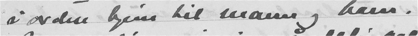i orden hjem til mann og barn.
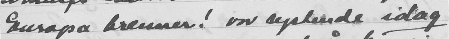Europa brenner! var rystende idag.
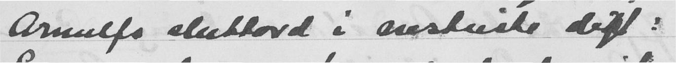Arnulfs sluttord i nestsiste døpt:
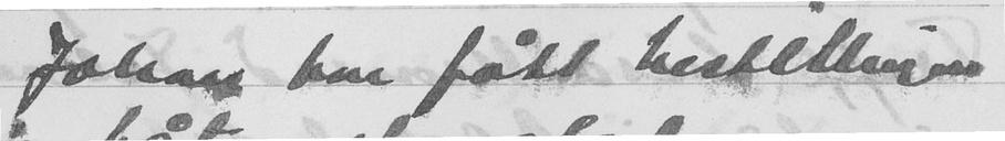Johan har fått bestillingen
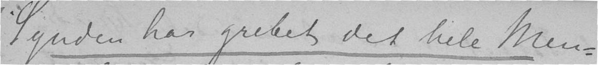Synden har grebet det hele Men-
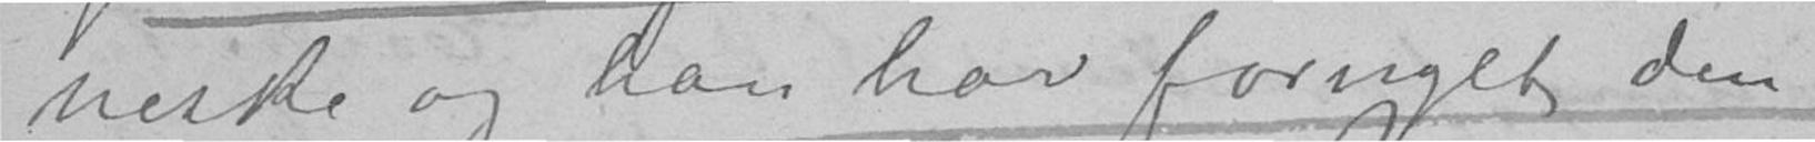neske og han har fornyet den i
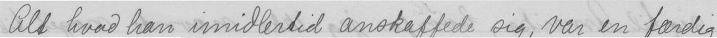Alt hvad han imidlertid anskaffede sig, var en færdig
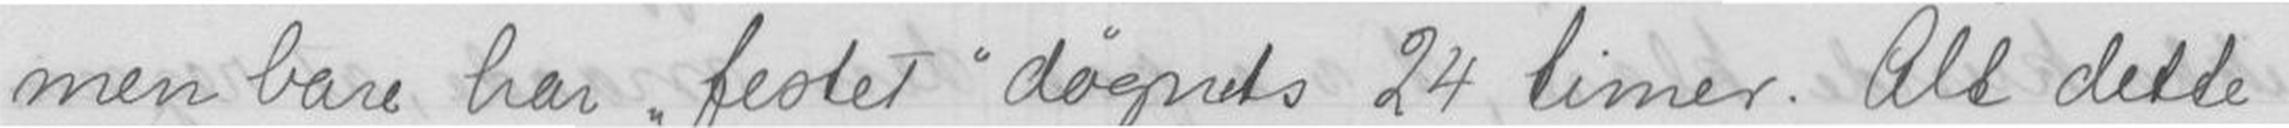men bare har festet døgnets 24 timer. Alt dette
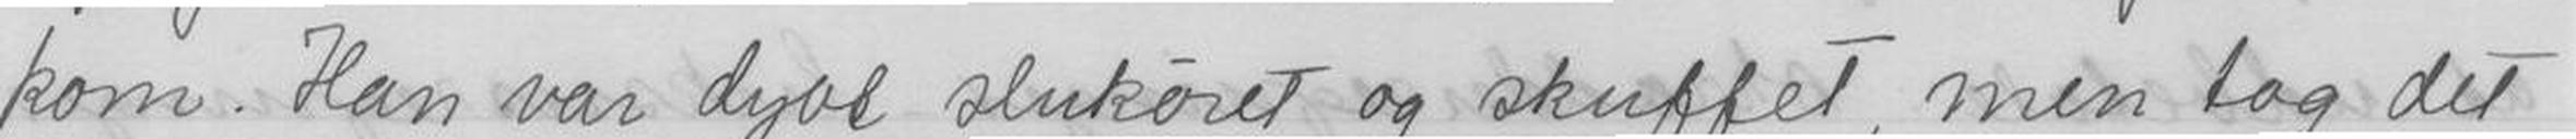kom. Han var dybt slukøret og skuffet, men tog det
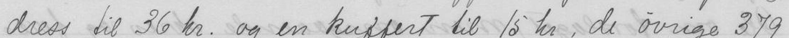dress til 36 kr. og en kuffert til 15 kr, de øvrige 379
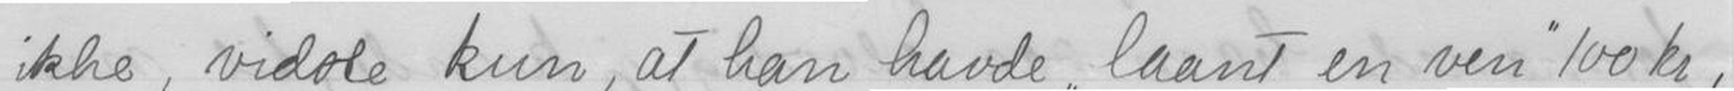ikke, vidste kun, at han havde laant en ven 100 kr,
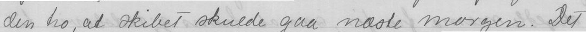den tro, at skibet skulde gaa næste morgen. Det
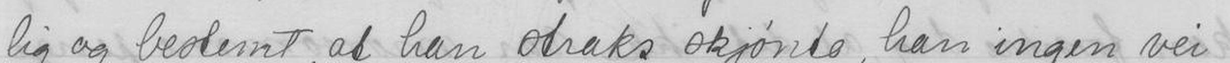lig og bestemt, at han straks skjønte, han ingen vei
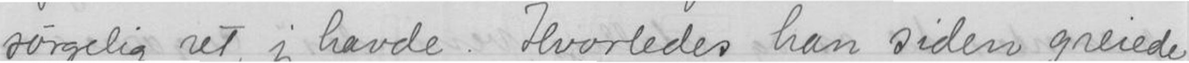sørgelig ret, jeg havde. Hvorledes han siden greiede
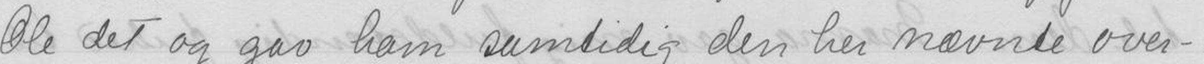Ole det og gav ham samtidig den her nævnte over-
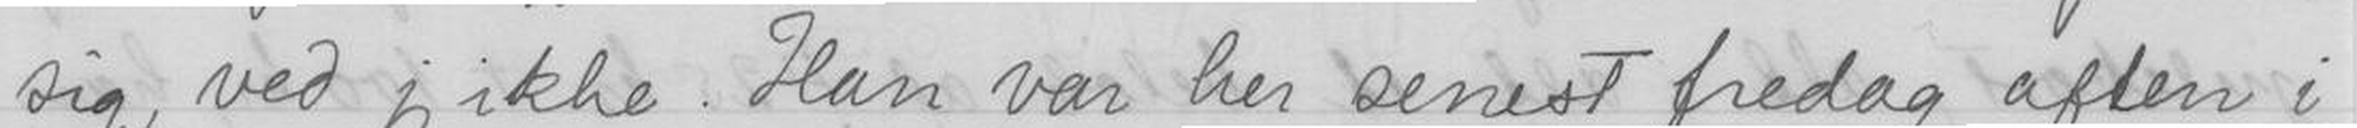sig, ved jeg ikke. Han var her senest fredag aften i
